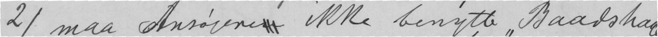2)maa Ansøgeren ikke benytte Baadshage-
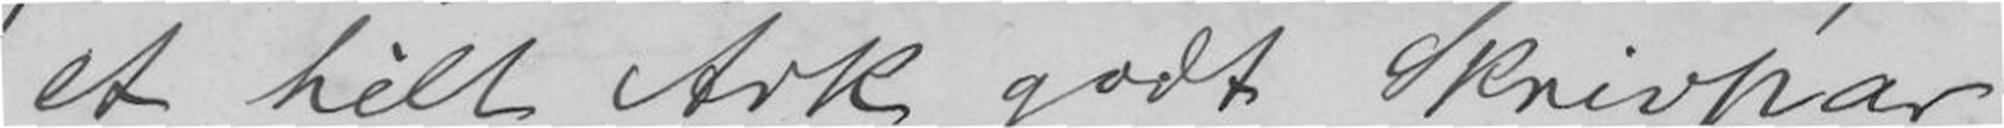et helt Ark godt Skrivpar.
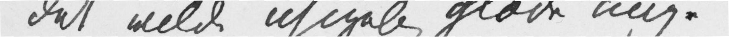det vilde usigelig glæde mig.
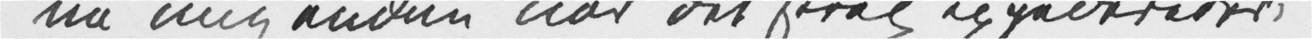nå mig endnu når det strax expederedes,
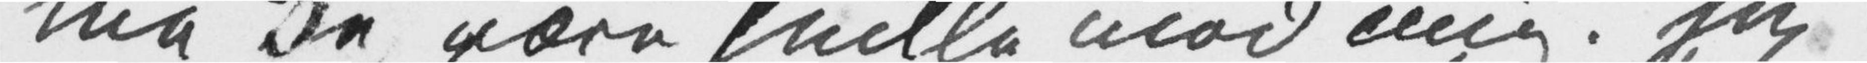må De være snille mod mig. Jeg
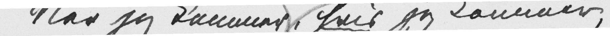Når jeg kommer, hvis jeg kommer,
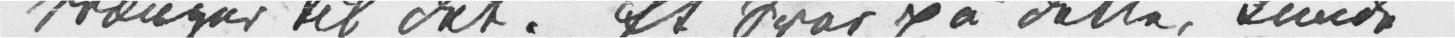trænger til det. Et Svar på dette, kunde
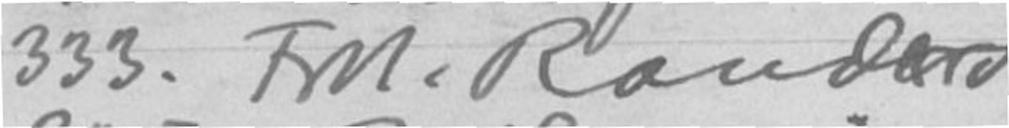333. Frk. Randers
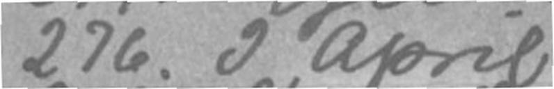276. I april
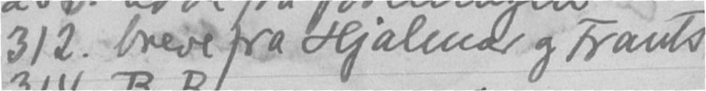312. breve fra Hjalmar og Frants
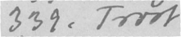339. Trøst
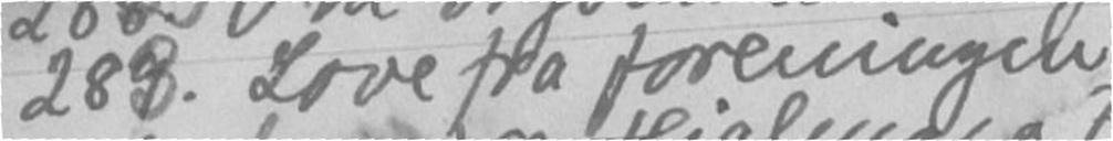283. Love fra foreningen
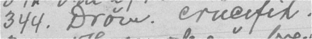344. Drøm. crucifix.
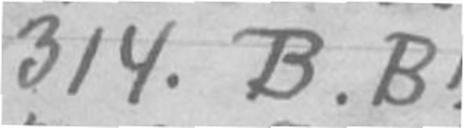314. B. B.
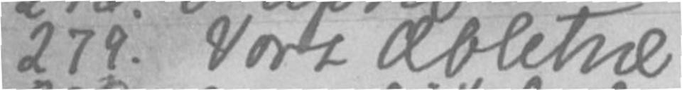279. Vort æbletre
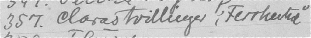357. Claras tvillinger; Ferohete"
